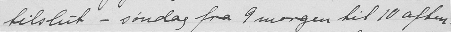tilslut - søndag fra 9 morgen til 10 aften -
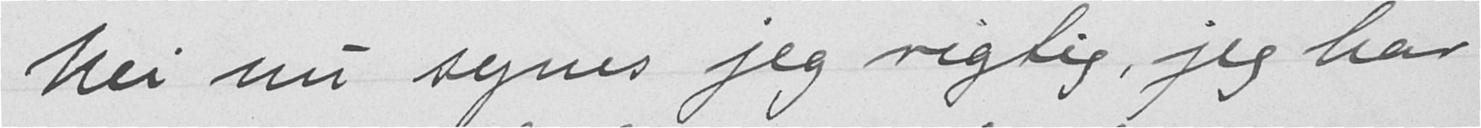Nei nu synes jeg rigtig, jeg har
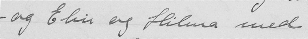- og Elin og Hilma med
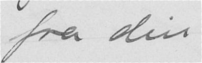fra din
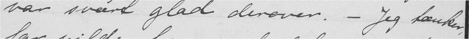var svært glad derover. - Jeg tænker,
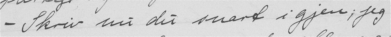- Skriv nu du snart igjen; jeg
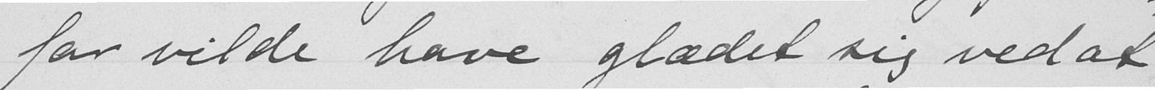far vilde have glædet sig ved at
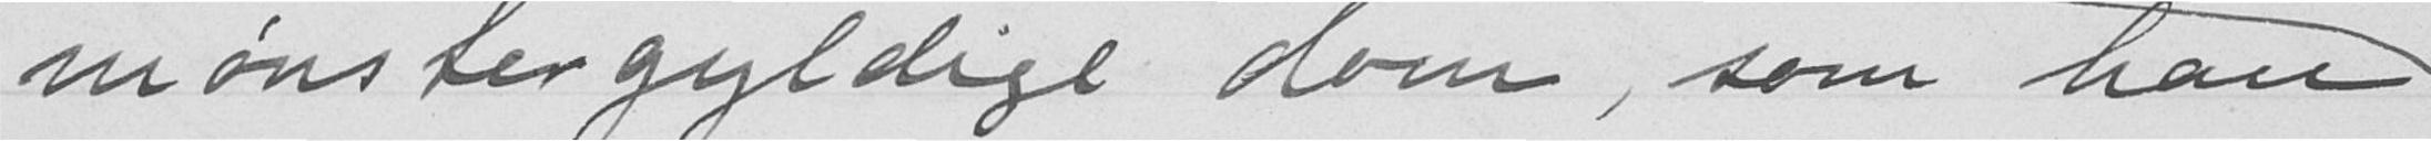mønstergyldige dom, som han
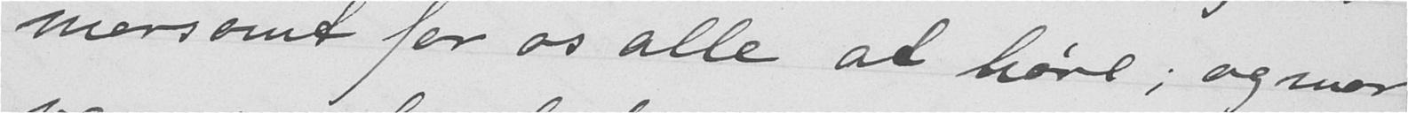morsomt for os alle at høre; og mor
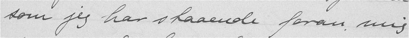som jeg har staaende foran mig
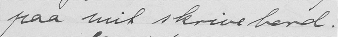paa mit skrivebord.
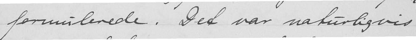formulerede. Det var naturligvis
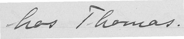hos Thomas.
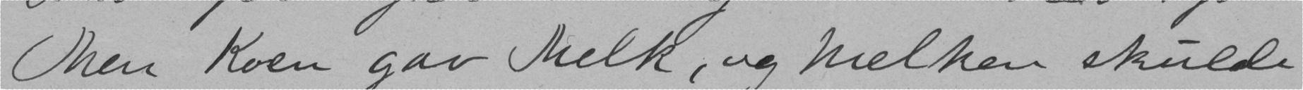Men Koen gav Melk, og melken skulde
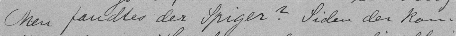Men fandtes der Spiger? Siden der kom
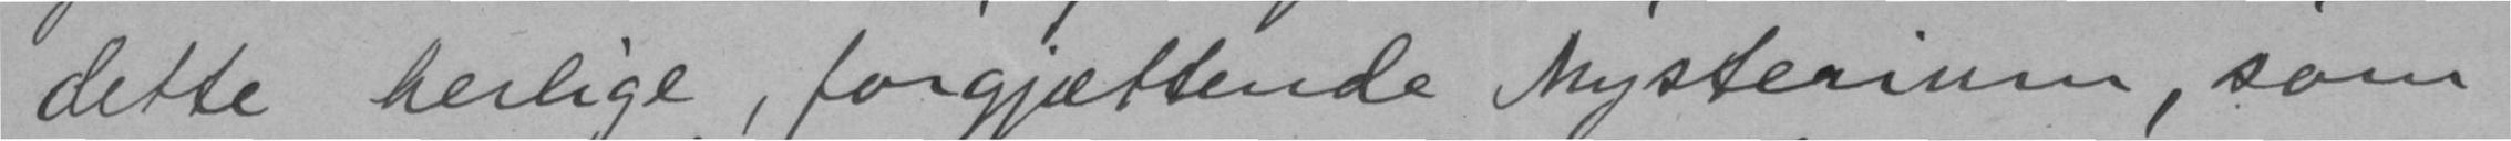dette herlige, forgjættende Mysterium, som
